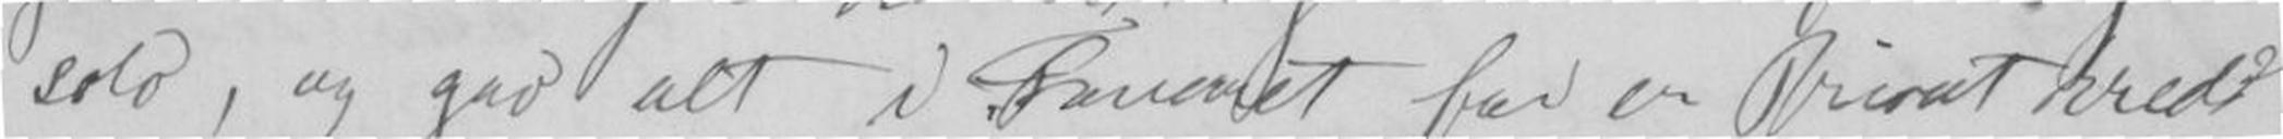solo, og gav alt i Concert for en Privat kreds
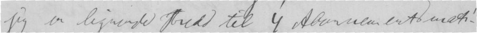jeg en lignende Kreds til 4 Abonnementmeti-
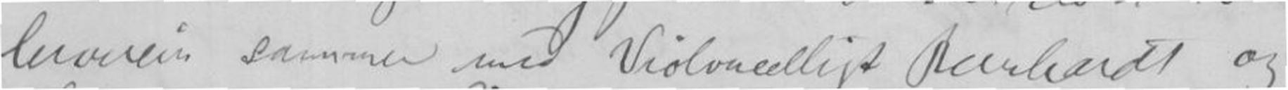lerverein sammen med Violoncellist Bernhardt og
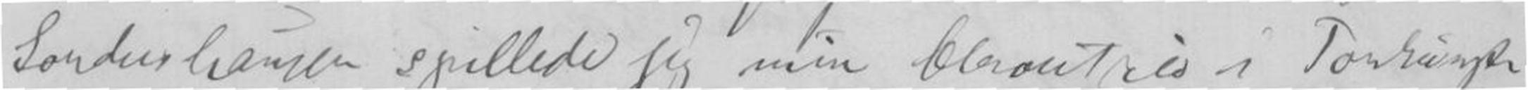Sonderhauen spillede jeg min Clavertro i Tonkünst-
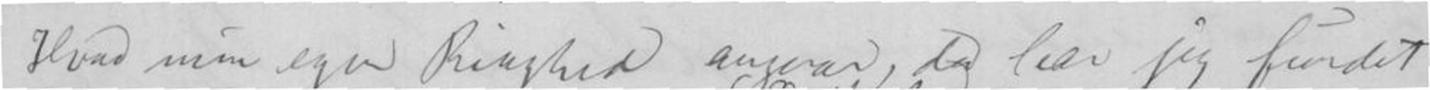Hvad min egen Ringhed angaar, da har jeg fundet
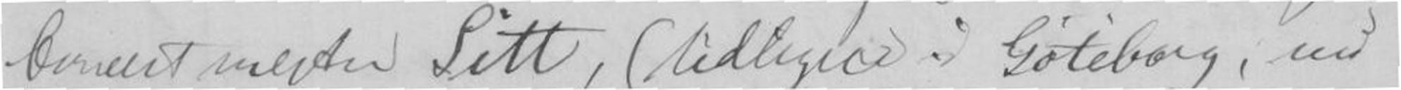Concertmester Sitt, (tidligere i Gøteborg, nu
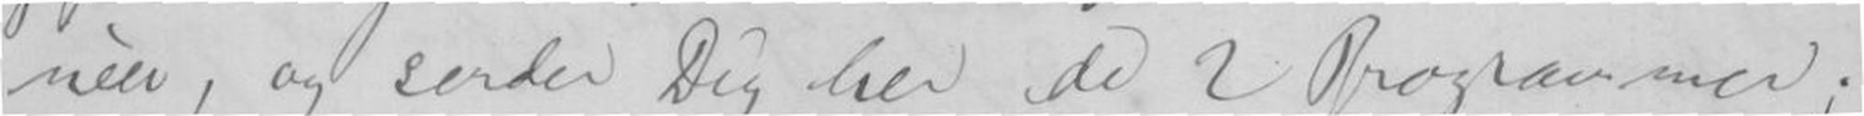néer, og sender Dig her de 2 Programmer;
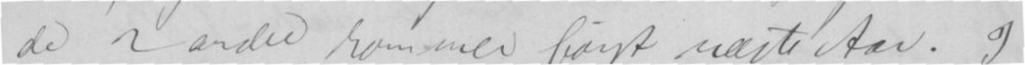de 2 andre kommer først næste Aar. I
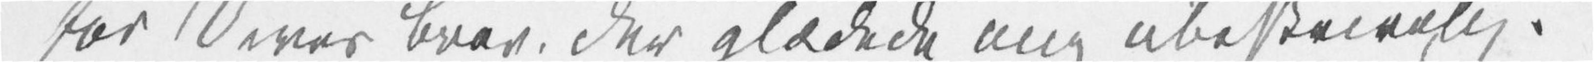for Deres Brev, der glædede mig ubeskrivelig.
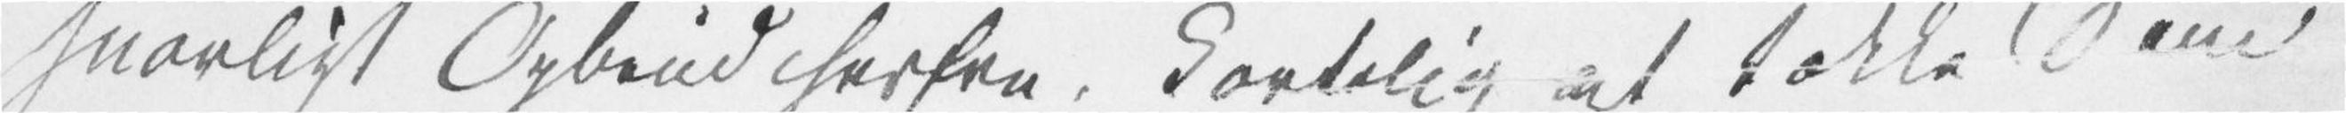snarligt Opbrud herfra, kortelig at takke Dem
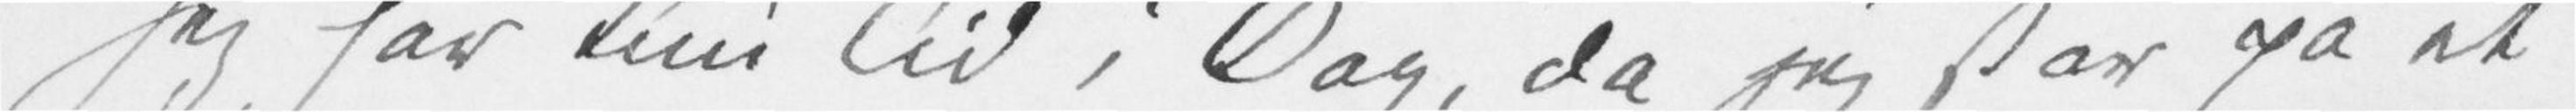Jeg har kun Tid i Dag, da jeg står på et
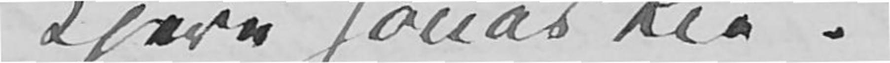Kjere Jonas Lie!
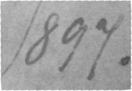1897.
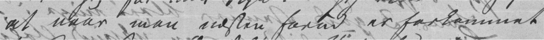at naar man næsten forud er forkommet
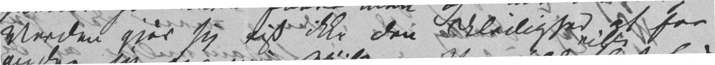Verden gjør sig vist ikke den Uleilighed at vilde for
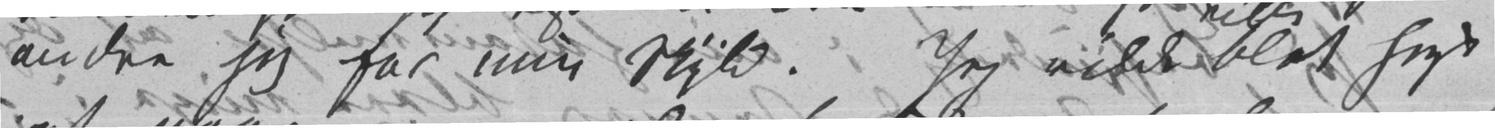andre sig for min Skyld. Jeg vilde blot sige
